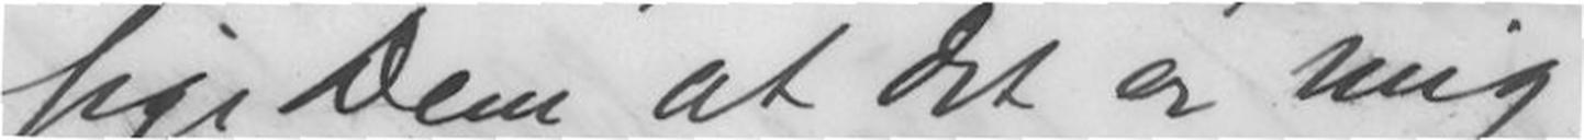sige Dem at det er mig
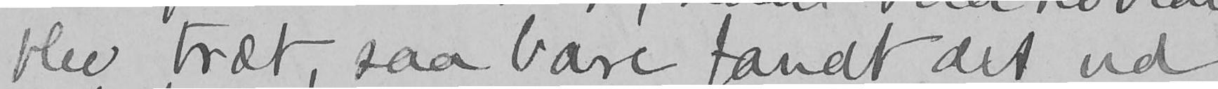blev træt, saa bare fandt det ud
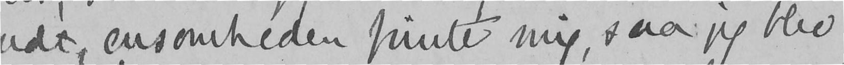ude, ensomheden pinte mig, saa jeg blev
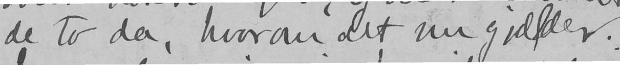de to da, hvorom det nu gjælder.
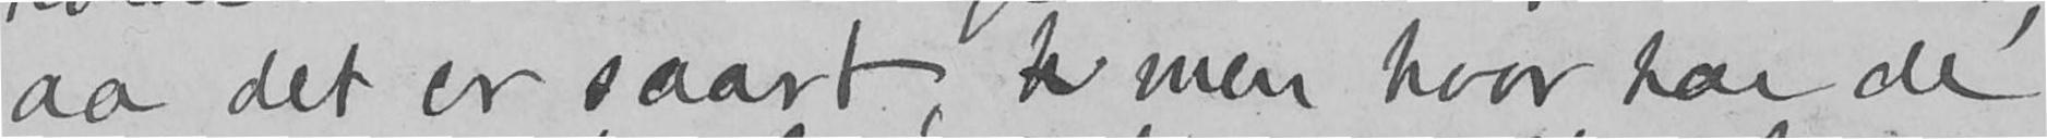aa det er saart, h men hvor har de
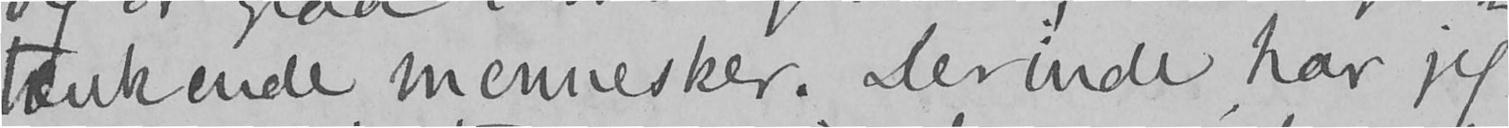tænkende mennesker. Derinde har jeg
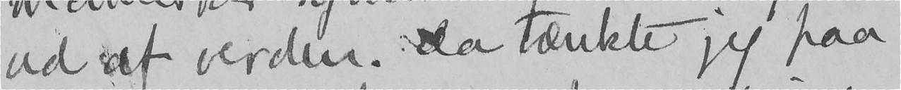ud af verden. Da tænkte jeg paa,
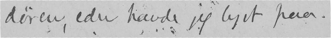døren, eder havde jeg lyst paa.
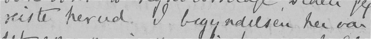reiste herud. I begyndelsen her var
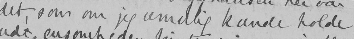det som om jeg umulig kunde holde
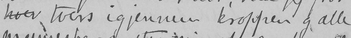hver tvers igjennem kroppen og alle
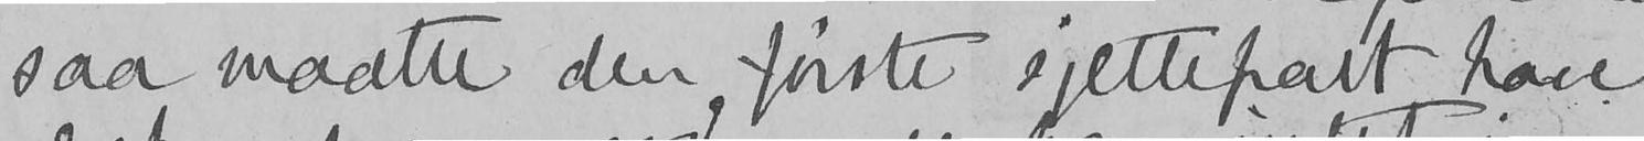saa maatte den første sjettepart have
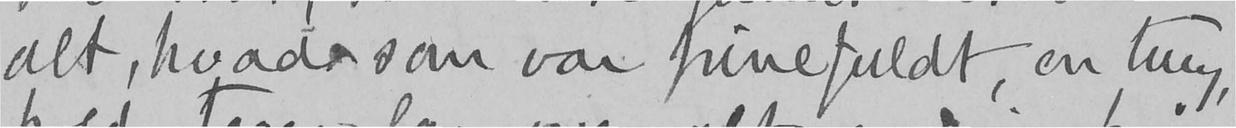alt, hvad som var pinefuldt, en tung,
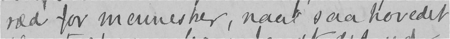ræd for mennesker, naar saa hovedet
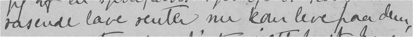rasende lave renter nu kan leve paa dem,
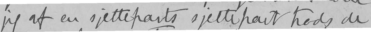jeg af en sjetteparts sjettepart trods de
